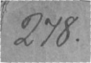278.
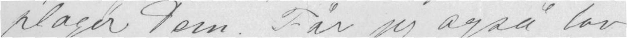plager Dem. Får jeg også lov
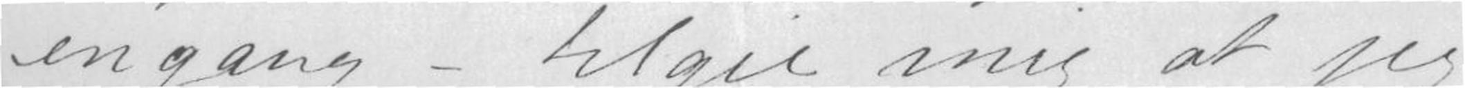engang - tilgie mig at jeg
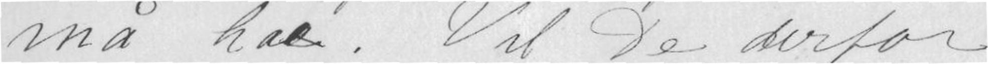må hae. Vil De derfor
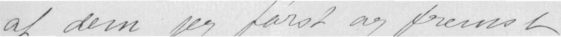af dem jeg først og fremst
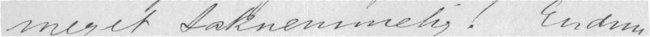meget taknemmelig! Endnu
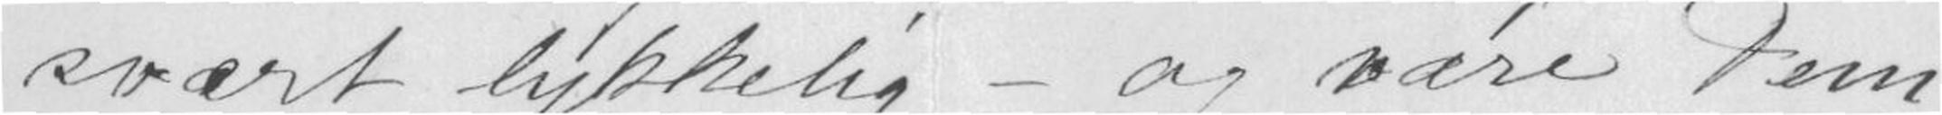svært lykkelig - og være Dem
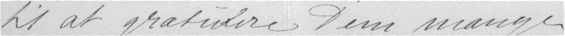til at gratulere Dem mange
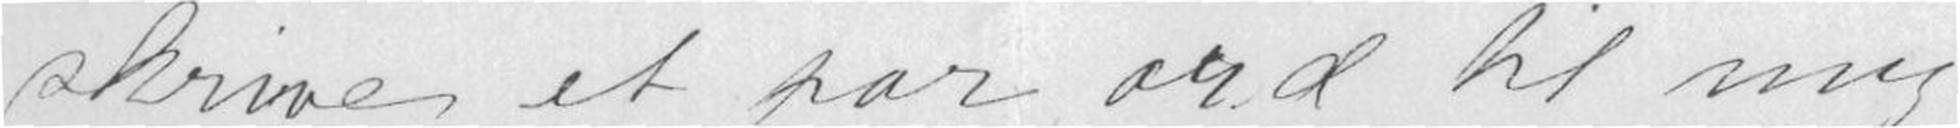skrive et par ord til mig,
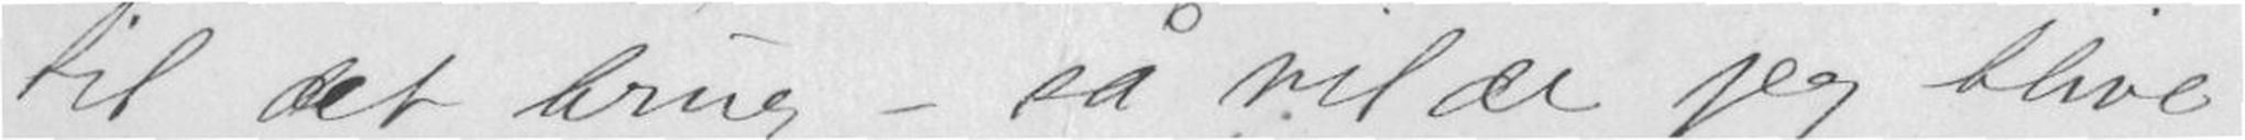til det brug - så vilde jeg blive
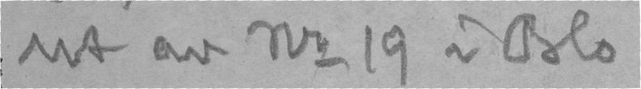ut av no 19 i Oslo
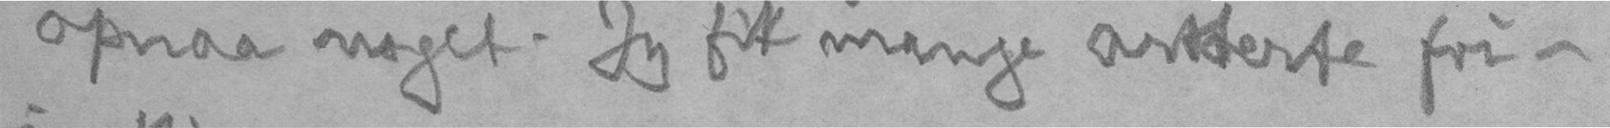opnaa noget. Jeg fik mange arsterte fri -
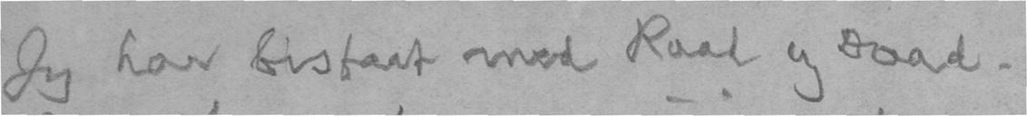Jeg har bistaat med Raad og Daad.
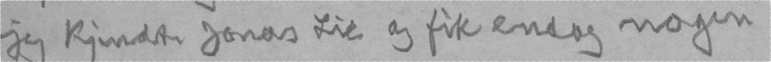jeg kjendte Jonas Lie og fik endog nogen
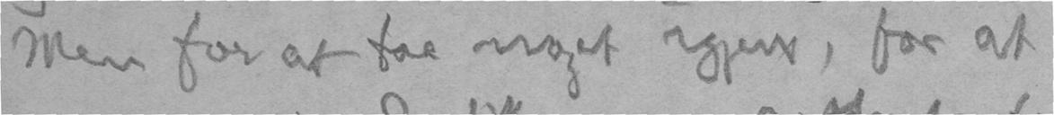Men for at faa noget igjen, for at
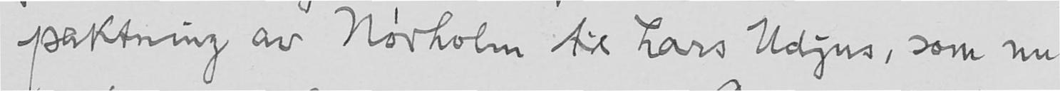paktning av Nørholm til Lars Udjus, som nu
